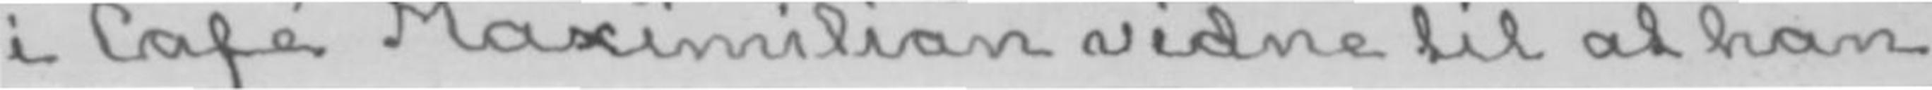i Café Maximilian vidne til at han
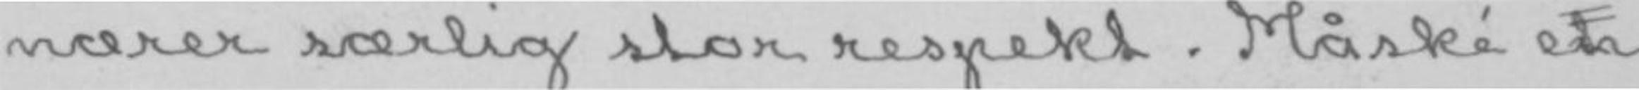nærer særlig stor respekt. Måské en
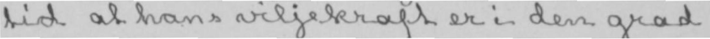tid at hans viljekraft er i den grad
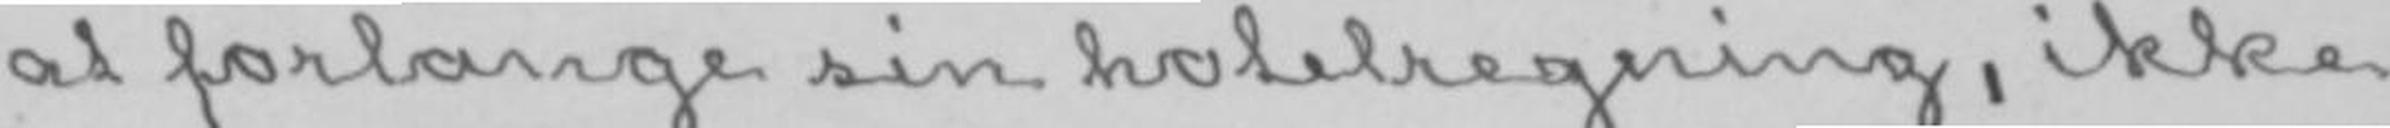at forlange sin hotelregning, ikke
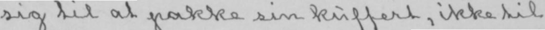sig til at pakke sin kuffert, ikke til
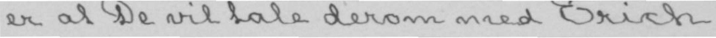er at De vil tale derom med Erich
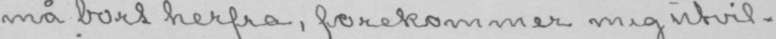må bort herfra, forekommer mig utvil-
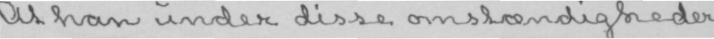At han under disse omstændigheder
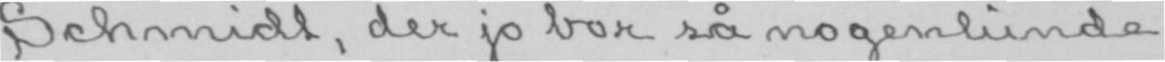Schmidt, der jo bor så nogenlunde
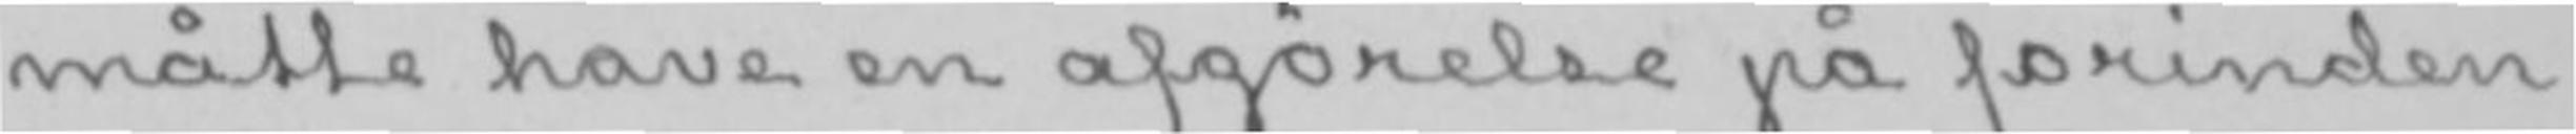måtte have en afgørelse på forinden
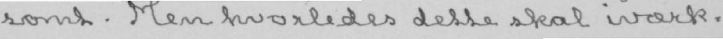somt. Men hvorledes dette skal iværk-
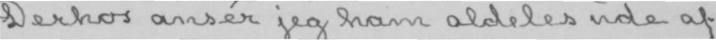Derhos ansér jeg ham aldeles ude af
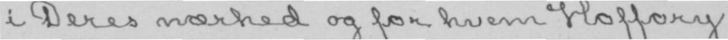i Deres nærhed og for hvem Hoffory
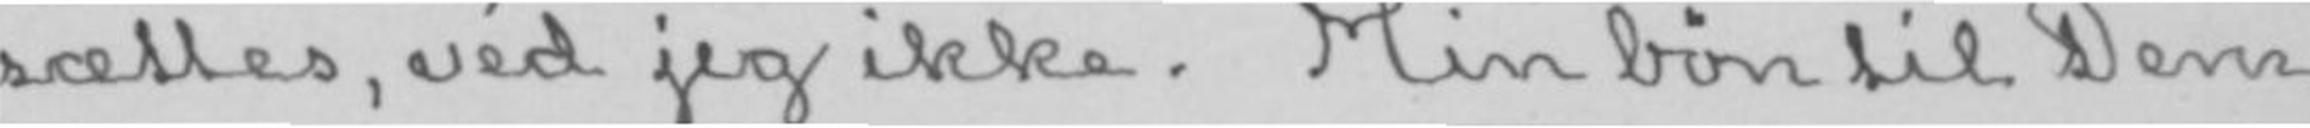sættes, véd jeg ikke. Min bøn til Dem
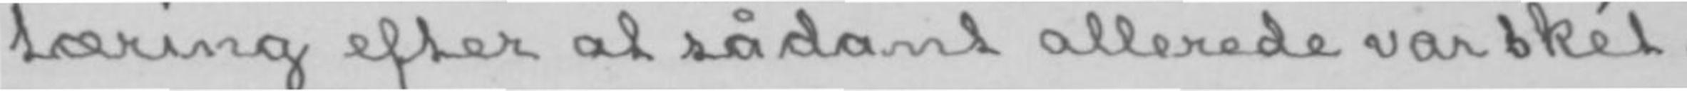tæring efter at sådant allerede var skét.
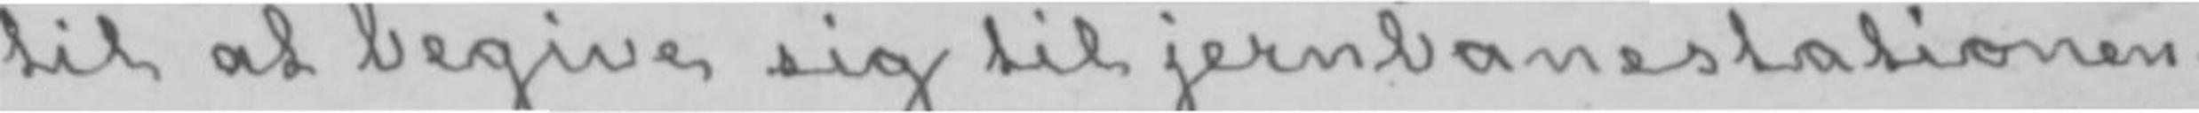til at begive sig til jernbanestationen.
